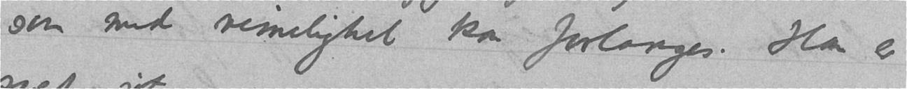som med rimelighet kan forlanges. Han er
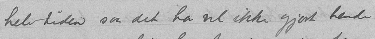hele tiden saa det har vel ikke gjort hende
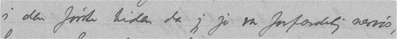i den første tiden da jeg jo var forfærdelig nervøs,
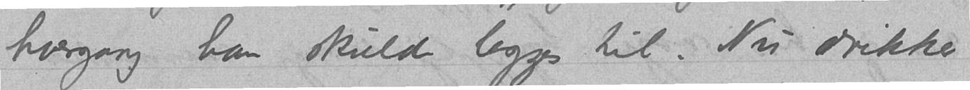hvergang han skulde legges til. Nu drikker
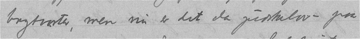brystvorter, men nu er det da gudskelov – paa
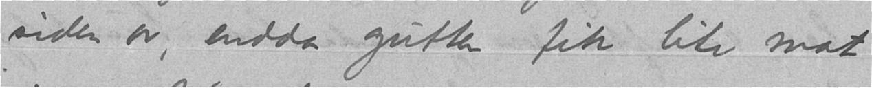siden av, endda gutten fik lite mat
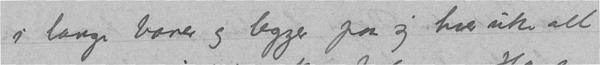i lange baner og legger paa sig hver uke alt
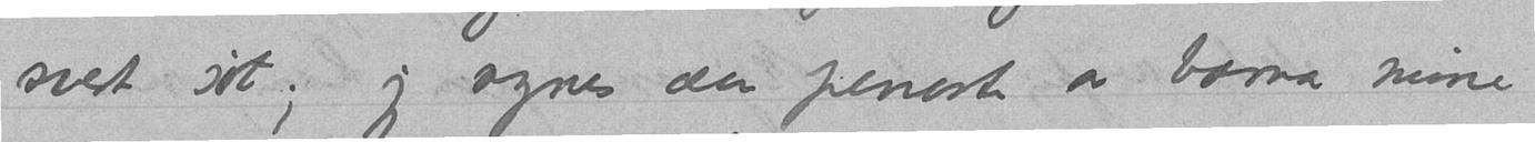svært søt; jeg synes den peneste av barna mine
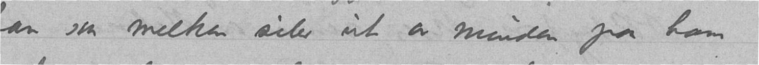han saa melken siler ut av munden paa ham
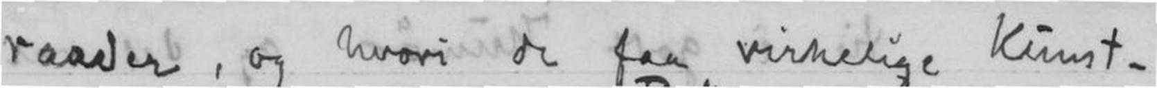raader, og hvori de faa virkelige Kunst-
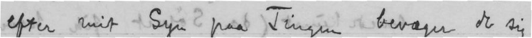efter mit Syn paa Tingene bevæger de sig
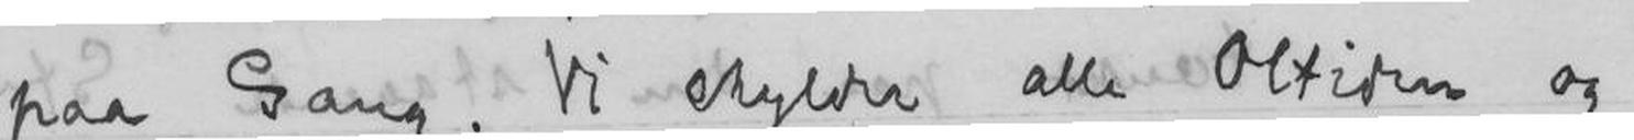paa Gang. Vi skylder alle Oldtiden og
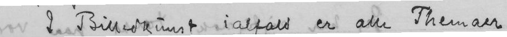I Billedkunst ialfald er alle Themaer
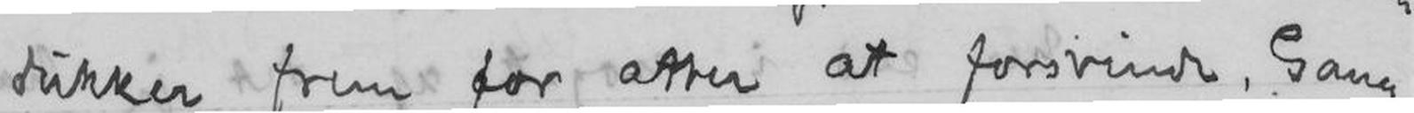dukker frem for atter at forsvinde, Gang
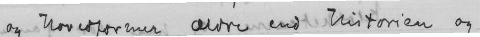og hovedformer ældre end Historien og
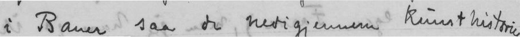i Baner saa de nedigjennem kunsthistorien
